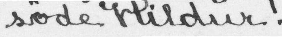søde Hildur!
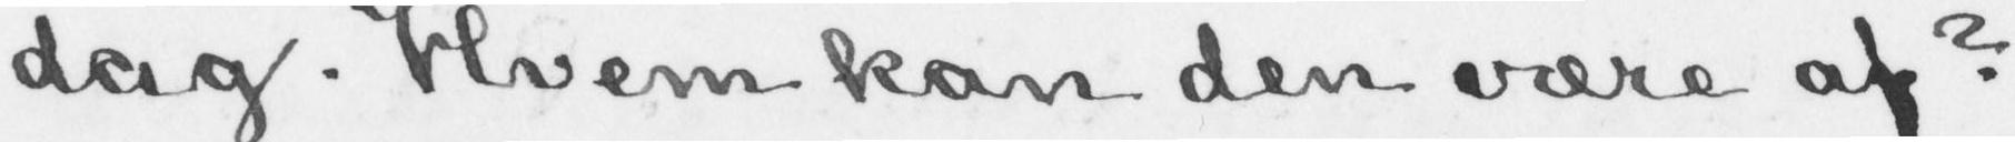dag. Hvem kan den være af?
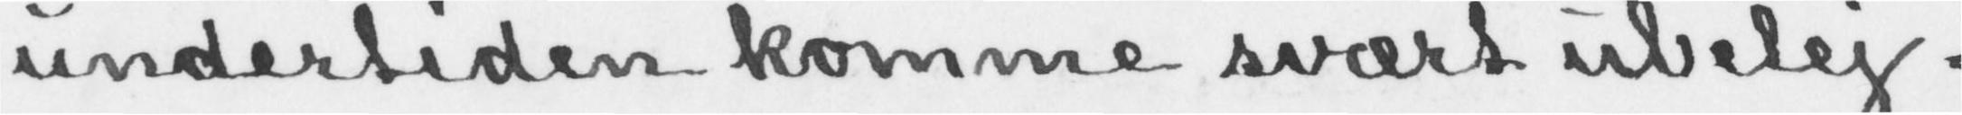undertiden komme svært ubelej-
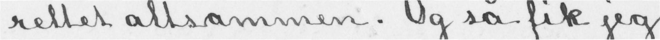rettet altsammen. Og så fik jeg
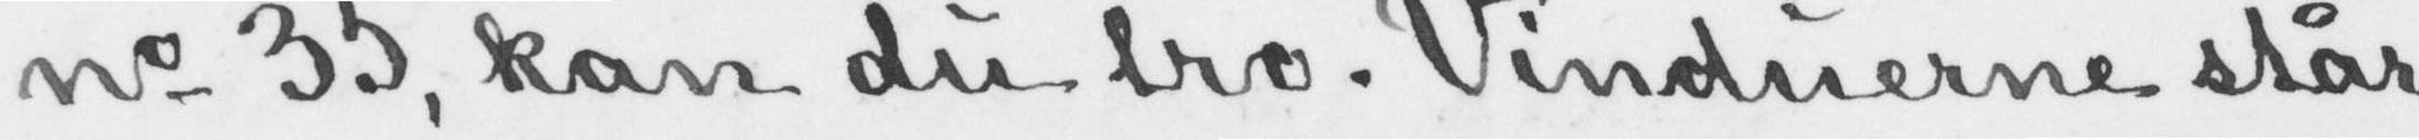no 35, kan du tro. Vinduerne står
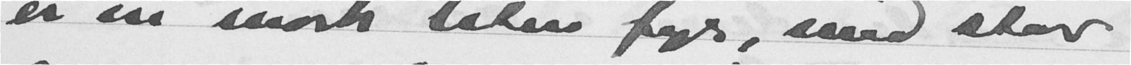er en mørk liten fyr, med stor
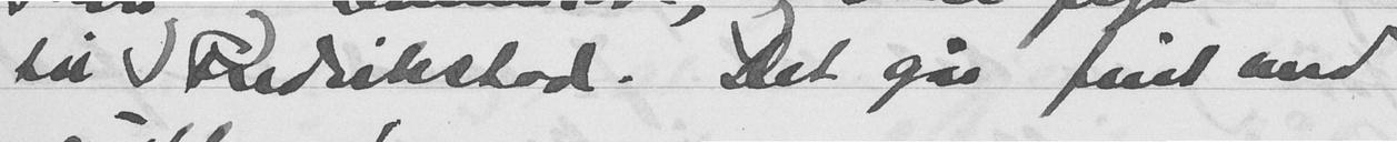til Fredrikstad. Det går fint med
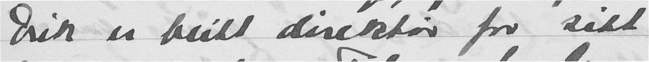Erik er blitt direktør for sitt
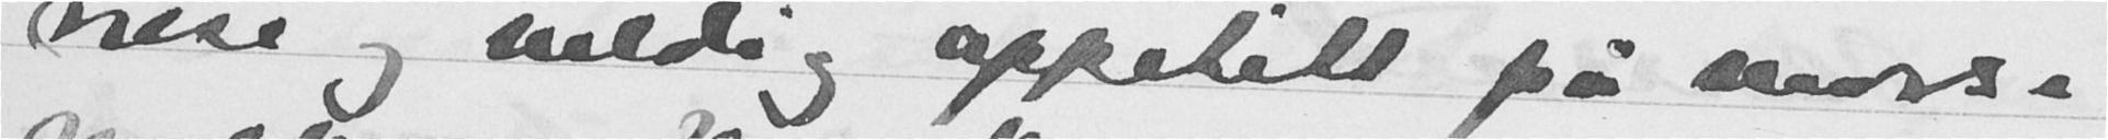nese og veldig appetitt på mors-
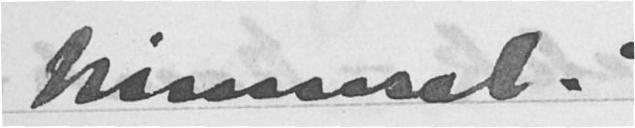himmel."
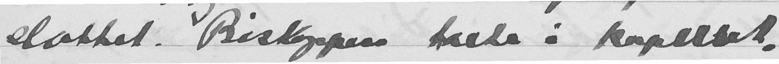slottet. Biskoppen talte i kapellet,
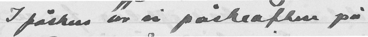I påsken var vi påskeaften på
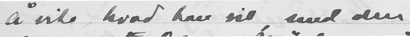å vite hvad han vil med den
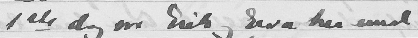1ste dag var Erik og Eva her med
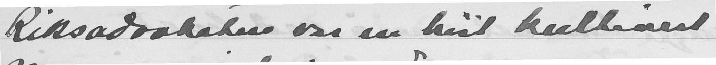Riksadvokaten var en høit kultivert
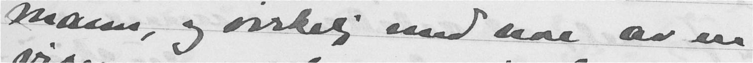mann, og virkelig med noe av en
